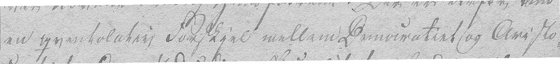en qvantitativ Forskjel mellem Democratiet og Aristo-
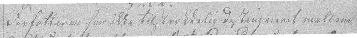Forfatteren har ikke tilstrækkelig distingveret mellem
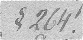§ 264
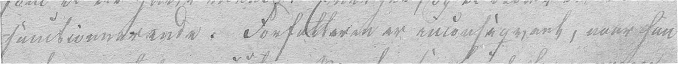functionerende. Forfatteren er inconseqvent, naar han
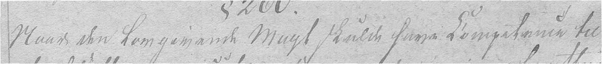Naar den Lovgivende Magt skulde have Competence til
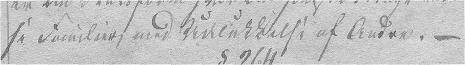se Familier med Udelukkelse af Andre. –
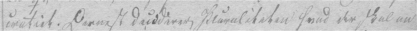cratiet. Dernæst deciderer Pluraliteten hvad der skal an-
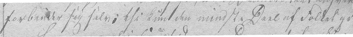forbinder sig selv; thi kun den mindste Deel af Folket gi-
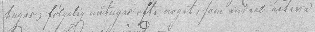tages; følgelig undtages ofte noget, som endeel active
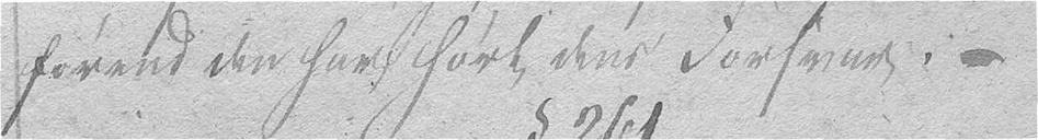førend den har hørt dens Forsvar. –
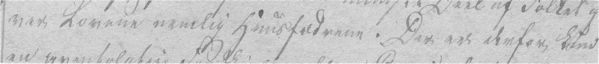ver Lovene nemlig Huusfædrene. Der er derfor kun
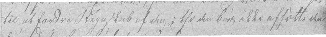til at fordre Regnskab af den; thi den bør ikke afsætte den
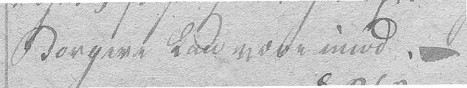Borgere kan være imod.–
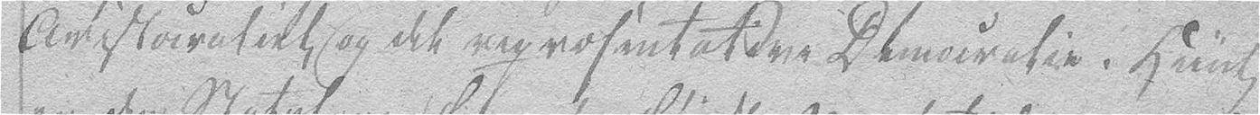Aristocratiet og det repræsentative Democratie. Hiint
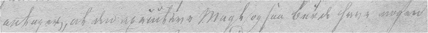antager, at den executive Magt ogsaa burde have nogen
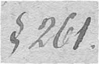§ 261.
